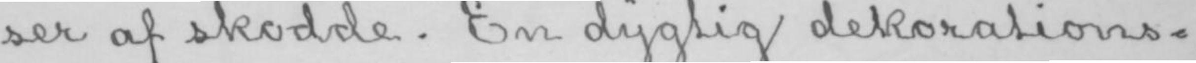ser af skodde. En dygtig dekorations-
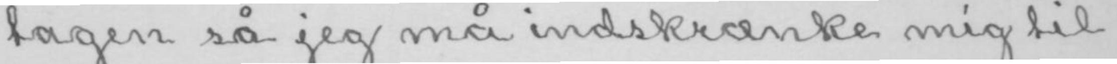tagen så jeg må indskrænke mig til
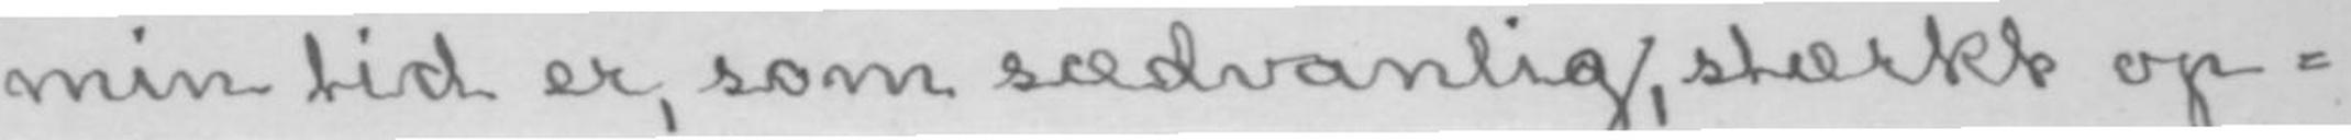min tid er, som sædvanlig, stærkt op-
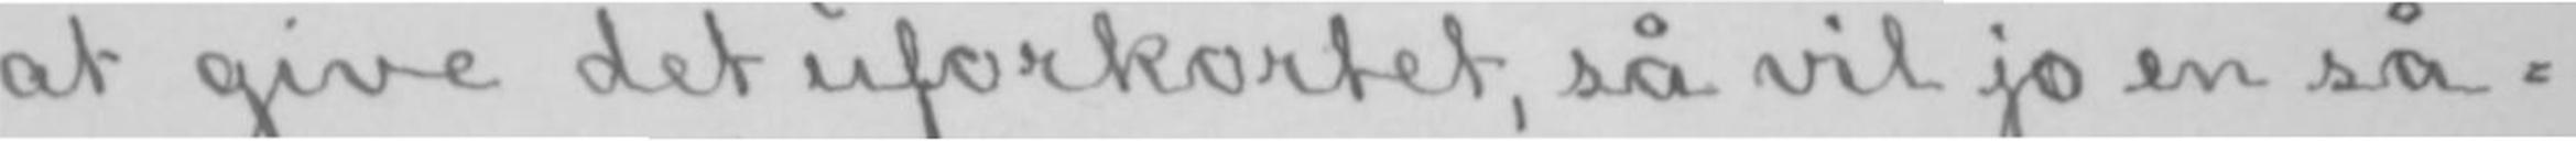at give det uforkortet, så vil jo en så-
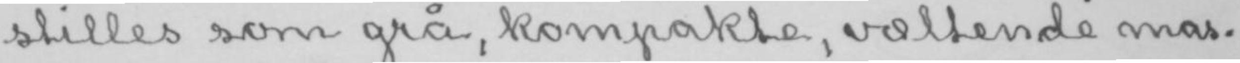stilles som grå, kompakte, væltende mas-
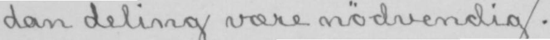dan deling være nødvendig.
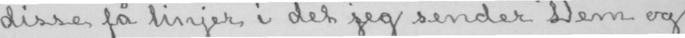disse få linjer i det sjeg sender Dem og
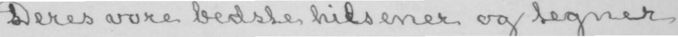Deres vore bedste hielsener og tegner
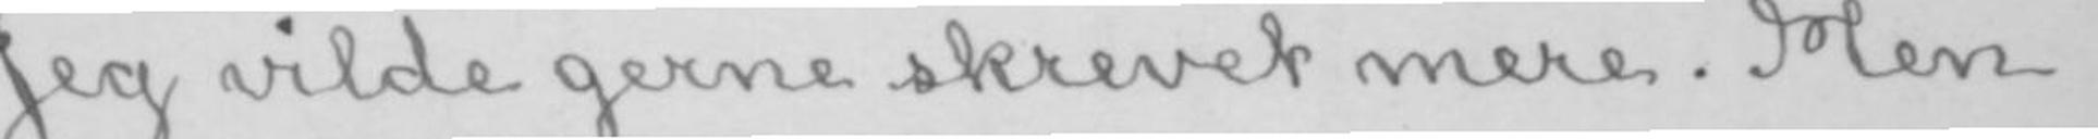Jeg vilde gerne skrevet mere. Men
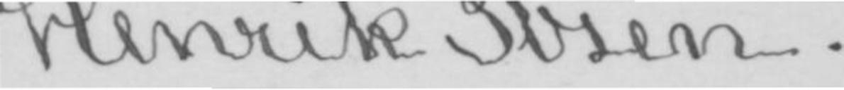Henrik Ibsen.
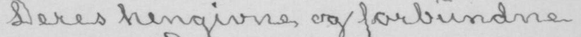Deres hengivne og forbundne
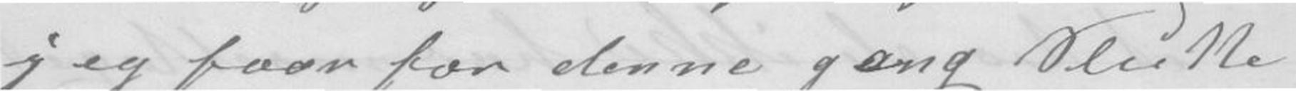jeg faar for denne gang Slutte
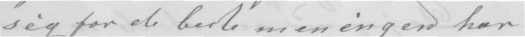sig for de beste menningen har
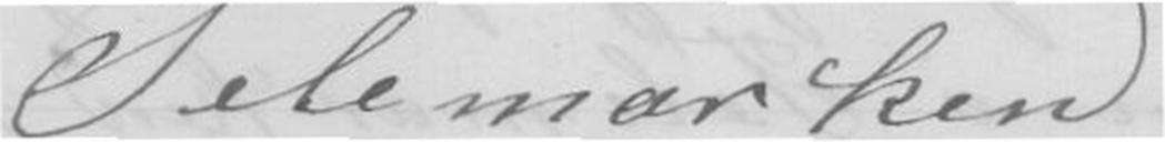Telemarken
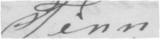Tinn
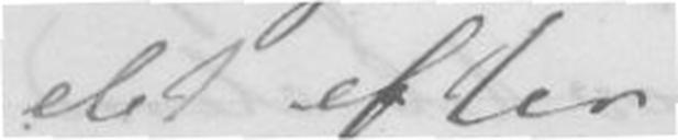de efter
